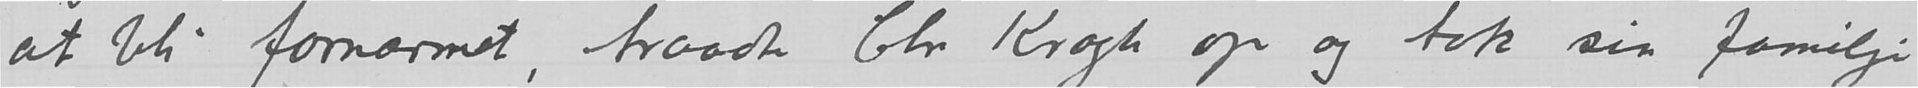at bli fornærmet, traadte Chr Krogh op og tok sin familje
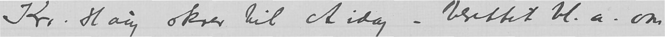Kri. Haug skrev til A idag - berettet bl.a. om
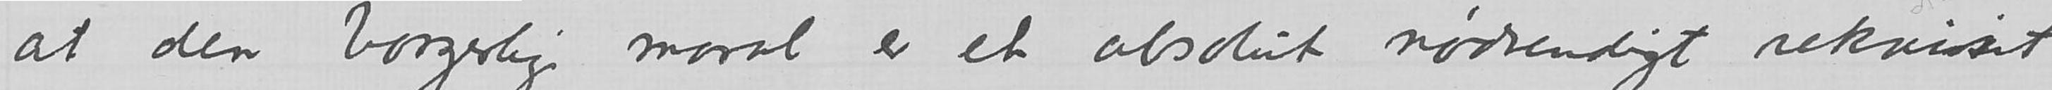at den borgerlige moral er et absolut nødvendigt rekvisit
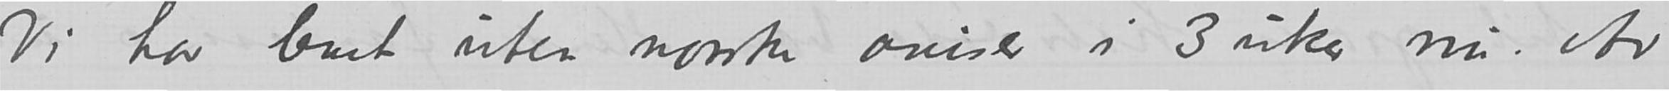Vi har levet uten norske aviser i 3 uker nu. Av
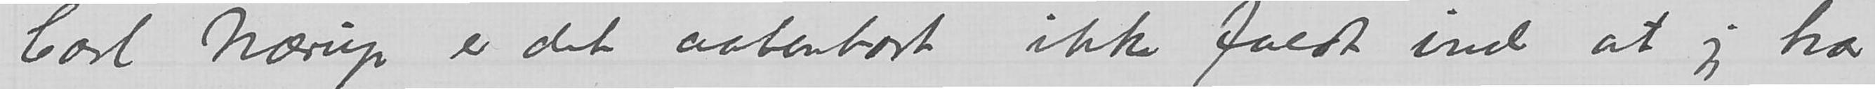Carl Nærup er det aabenbart ikke faldt ind at jeg har
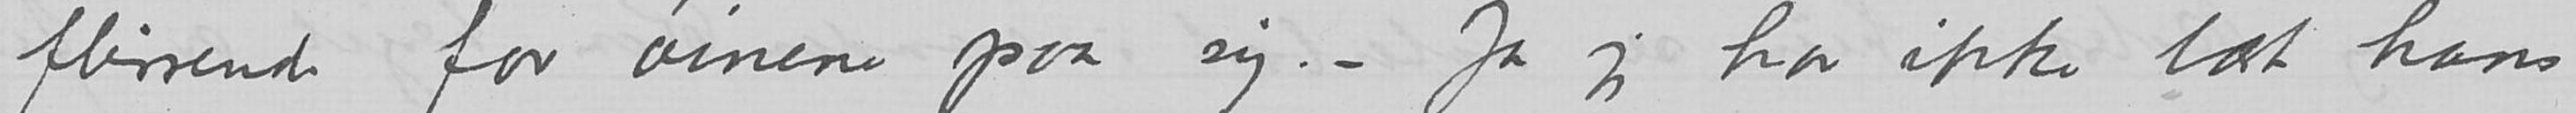flirrende for øinene paa sig. - Ja jeg har ikke læst hans
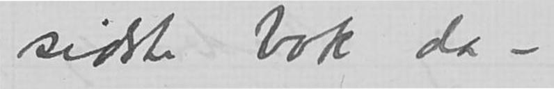sidste bok da -.
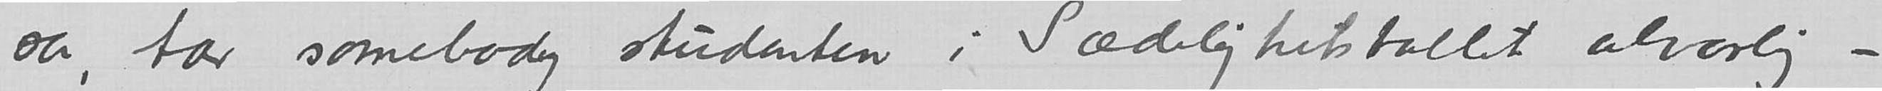tar somebody studenten i Sædelighetstallet alvorlig -
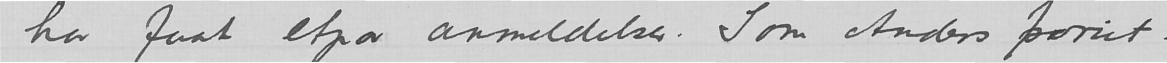Jeg har faat etpar anmeldelser. Som Anders forutsaa-
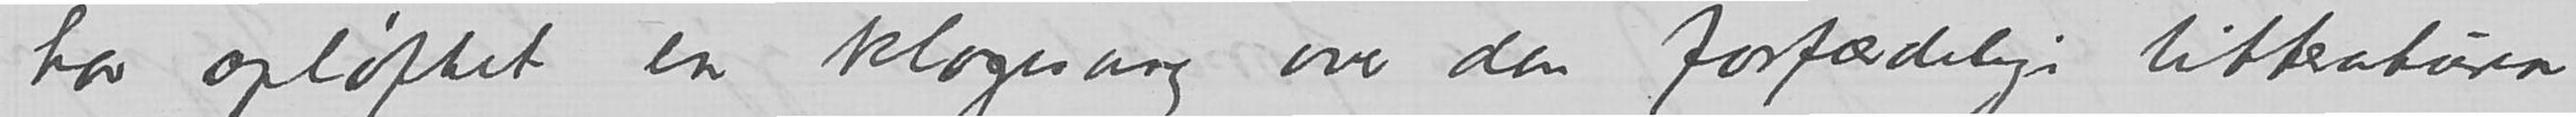har opløftet en klagesang over den forfærdelige litteraturen
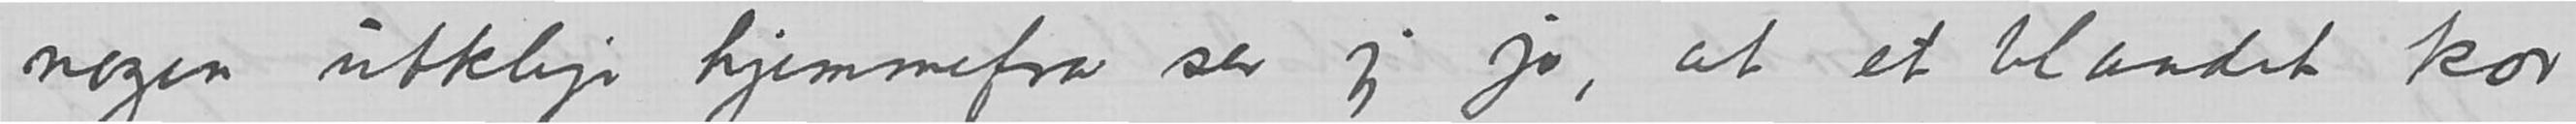nogen utklip hjemmefra ser jeg jo, at et blandet kor
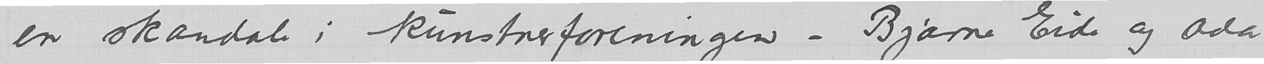en skandale i kunstnerforeningen - Bjarne Eide og Oda
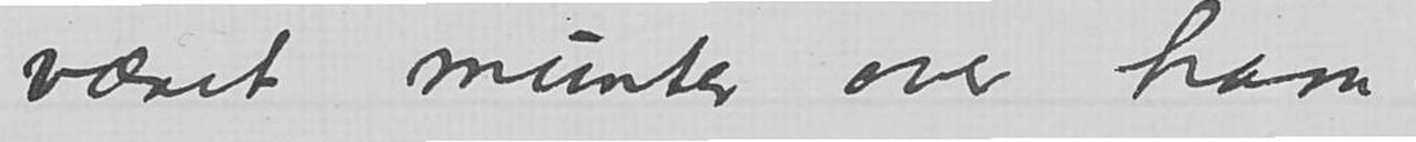været munter over ham.
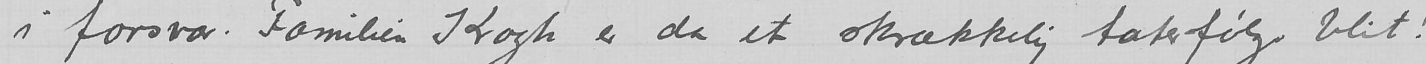i forsvar. Familien Krogh er da et skrækkelig taterfølge blit!
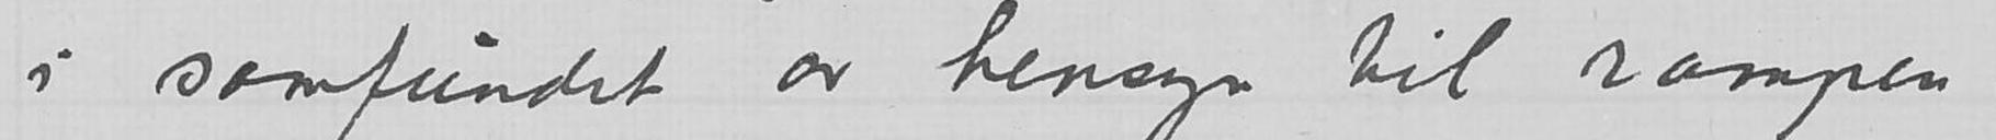i samfundet av hensyn til rampen. -
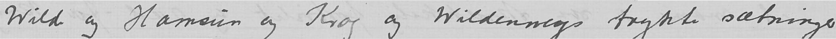Wilde og Hamsun og Krog og Wildenweys trykte sætninger
